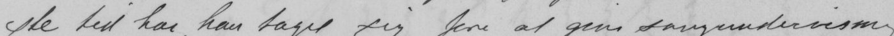ste tid har, han taget sig fore at give sangundervisning
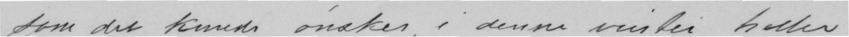som det kunde ønskes, i denne vinter heller.
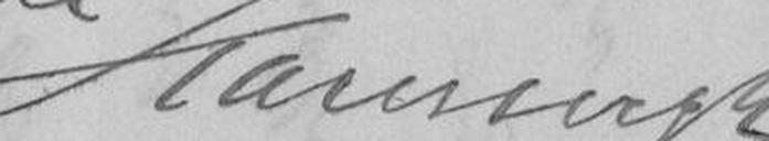Karl Berg
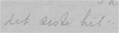det siste hit.
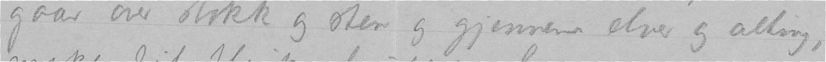gaar over stokk og sten og gjennem elver og alting,
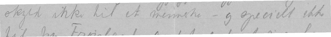skyld ikke til et menneske – og specielt ikke
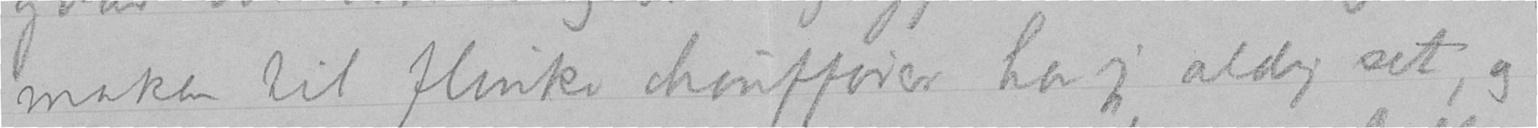maken til flinke chauffører har jeg aldrig set, og
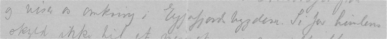og viser os omkring i Eyjafjordsbygdene. Si for himlens
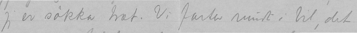jeg er søkka træt. Vi farter rundt i bil, det
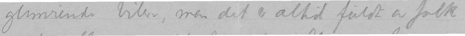glimrende biler, men det er altid fuldt av folk
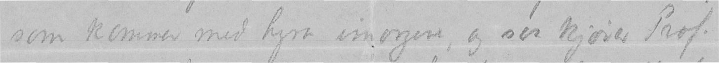som kommer med hyre imorgen, og saa kjører Prof.
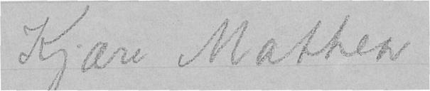Kjære Mathea
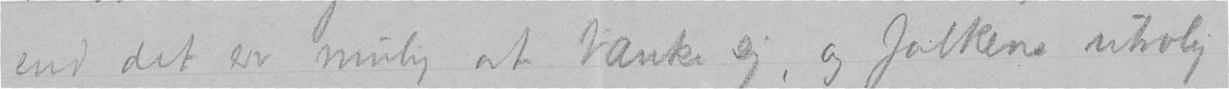end det er mulig at tænke sig, og folkene utrolig
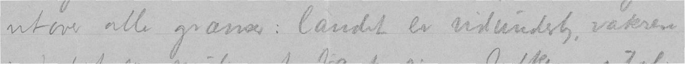utover alle grænser: landet er viderunderlig, vakrere
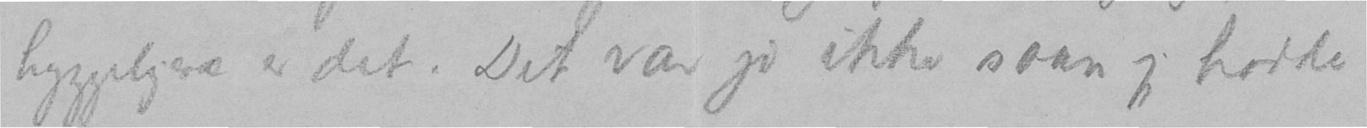hyggeligere er det. Det var jo ikke saan jeg hadde
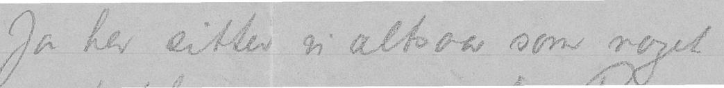Ja her sitter vi altsaa som noget
